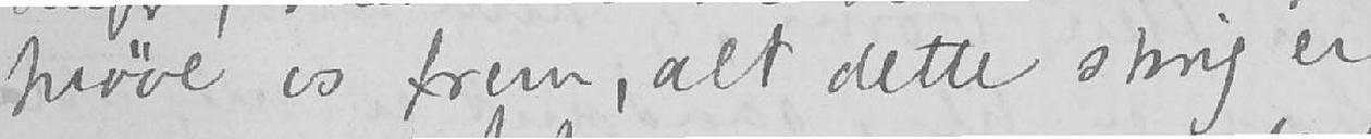prøve os frem, alt dette skrig er
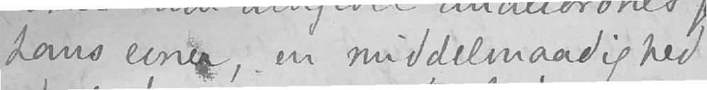hans evner, en middelmaadighed
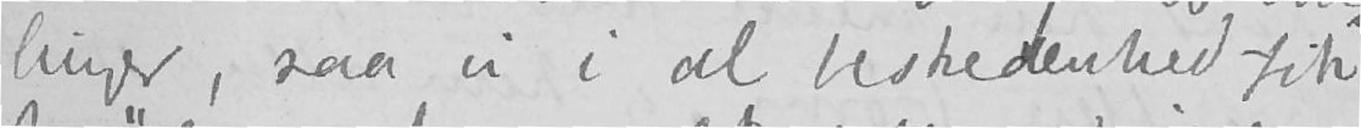linger, saa vi i al beskedenhed fik
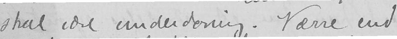skal være underdanig. Værre end
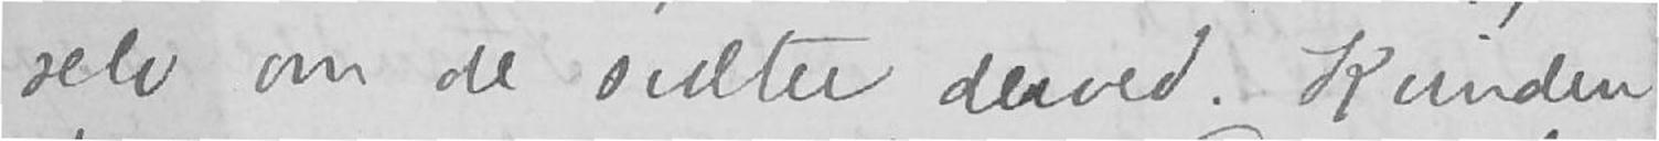selv om de sulter derved. Kvinden
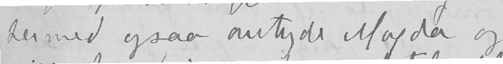hermed ogsaa antyde Magda og
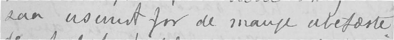saa usundt for de mange ubefæste-
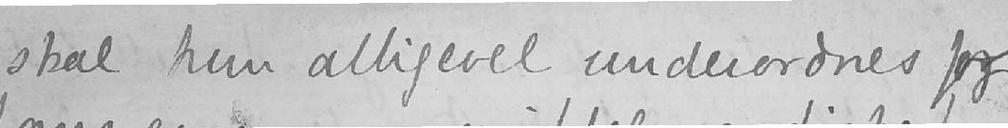skal hun alligevel underordnes for
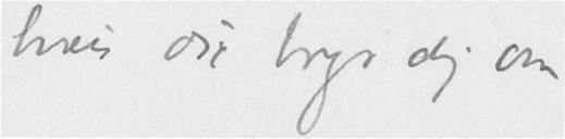hvis du bryr dig om – .
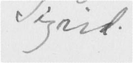Sigrid.
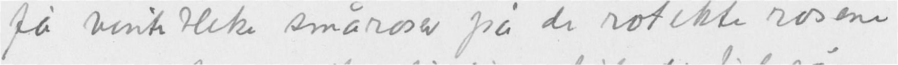få vinterbleke småroser på de rotekte rosene
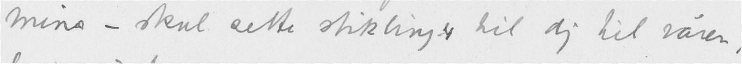mine – skal sette stiklinger til dig til våren
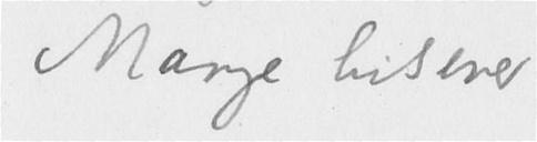Mange hilsener
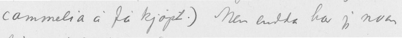cammelia å få kjøpt.) Men endda har jeg noen
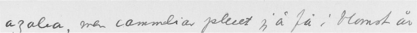azalea, men cammeliar pleiet jeg å få i blomst år
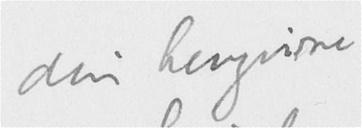din hengivne
